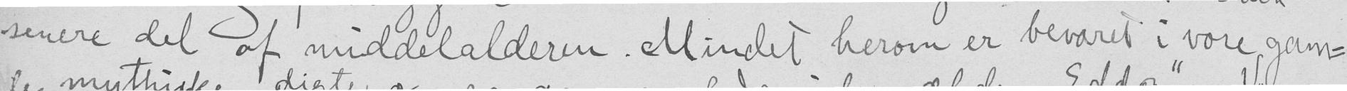senere del af middelalderen. Mindet herom er bevaret i vore gam-
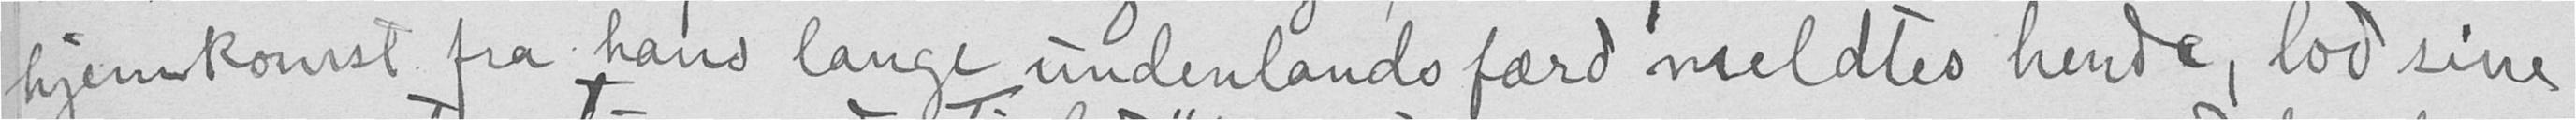hjemkomst fra hans lange undenlands færd meldtes hende, lod sine
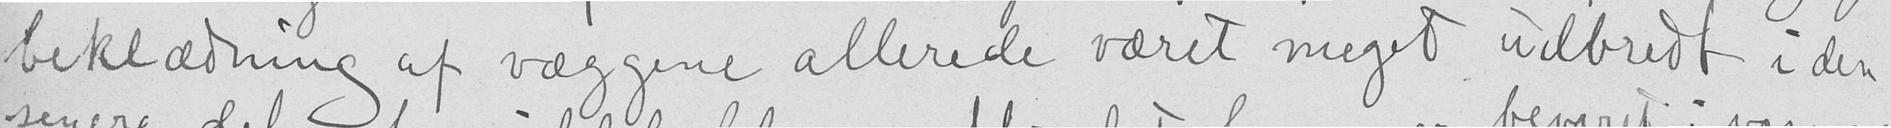beklædning af væggene allerede været meget udbredt i den
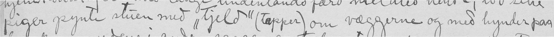piger pynte stuen med "tjeld (tæpper) om væggerne og med hynder paa
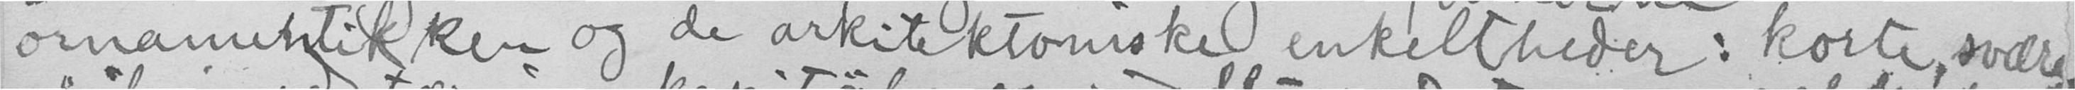ornametikken og de arkitektoniske enkeltheder: korte sværd
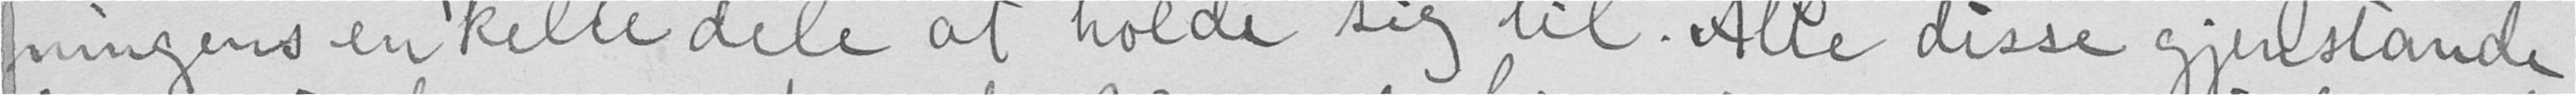ningens enkelte dele at holde sig til. Alle disse gjenstande
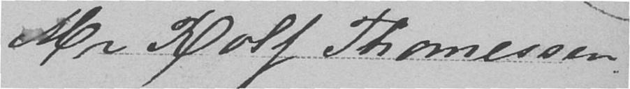Mr Rolf Thomessen
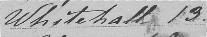Whitehall 13.
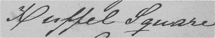Ruffel Square
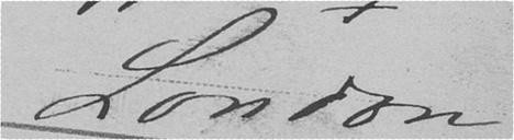London
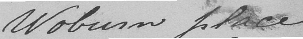Woburn place
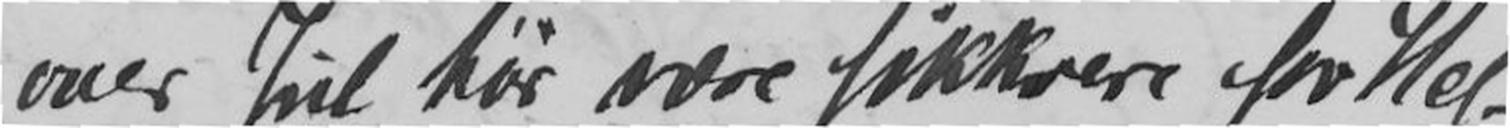over Jul tør være sikkrere for Hel-
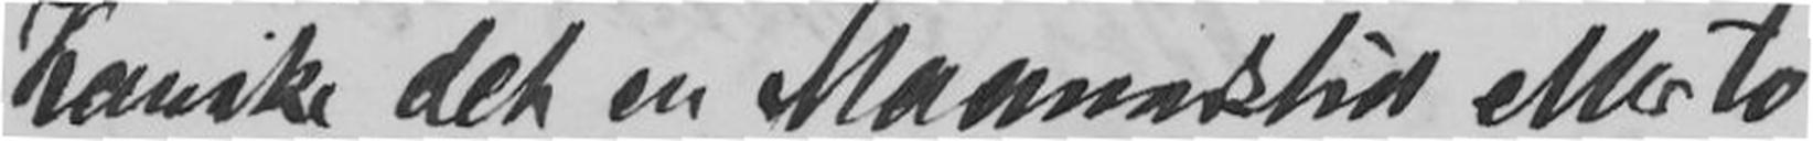Kanske det en Maanedstid eller to
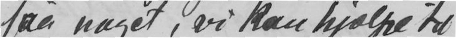saa noget, vi kan hjælpe til
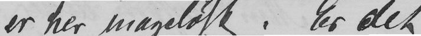er her mageløst. Er det
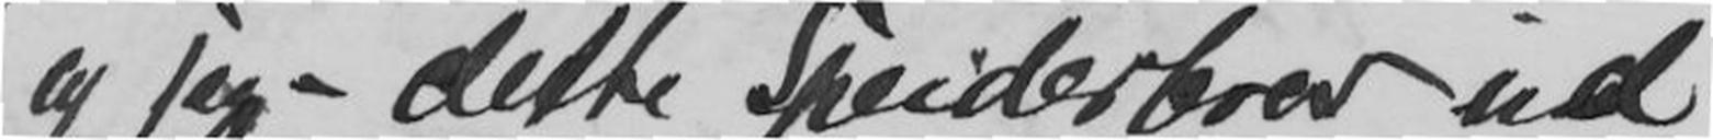og jeg - dette Speiderbrev ud
